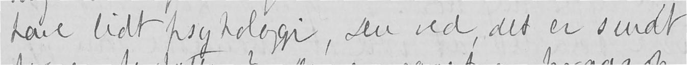have lidt psykologi, Du ved, det er sundt
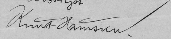Knut Hamsun.
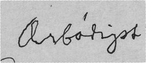Ærbødigst
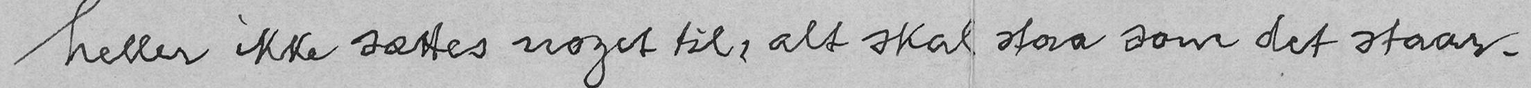heller ikke sættes noget til, alt skal staa som det staar.
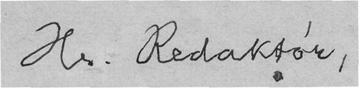Hr. Redaktør,
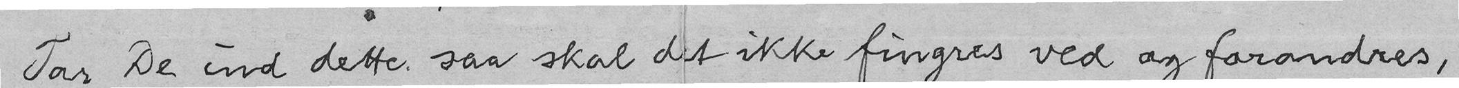Tar De ind dette, saa skal det ikke fingres ved og forandres,
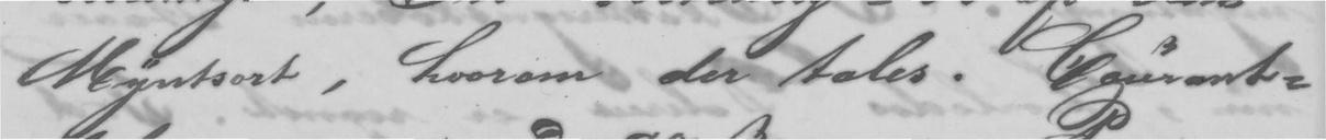Myntsort, hvorom der tales. Courant-
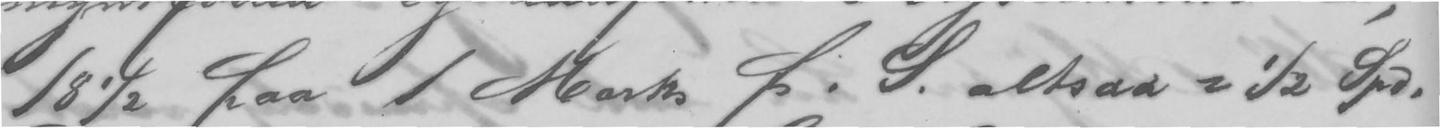18½ paa 1 Mark p. S. altsaa = ½ Spd.
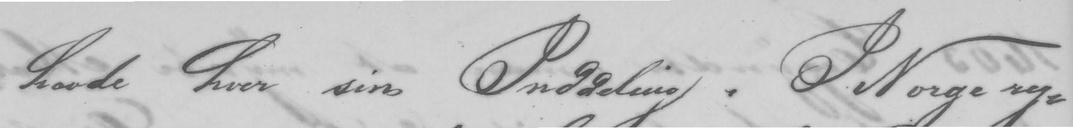havde hver sin Inddeling. I Norge reg-
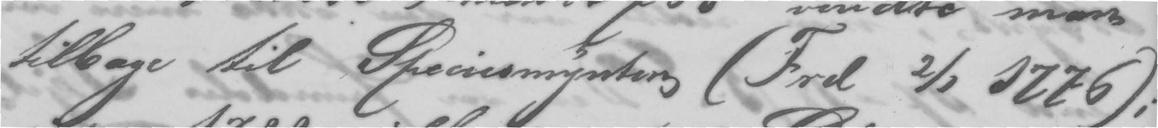tilbage til Speciesmynten (Frd 2/1 1776):
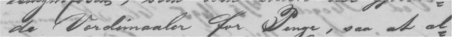de Værdimaaler for Penge, saa at al-
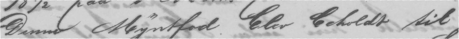Denne Myntfod blev beholdt til
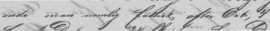nede man nemlig faktisk efter Ort, 4
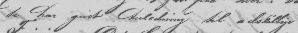te har givet Anledning til adskillige
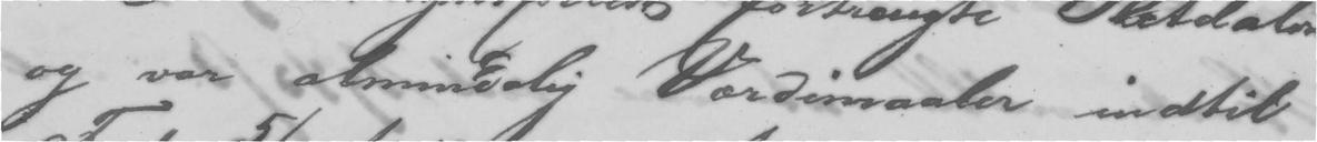og var almindelig Værdimaaler indtil
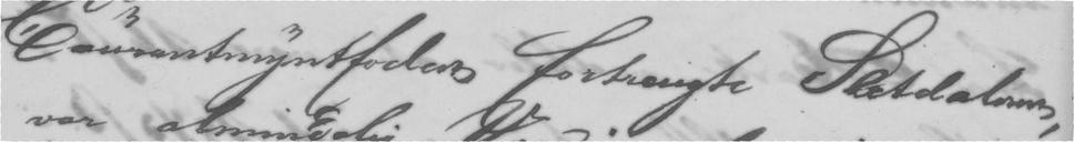Courantmyntfoden fortengte Sletdaleren,
